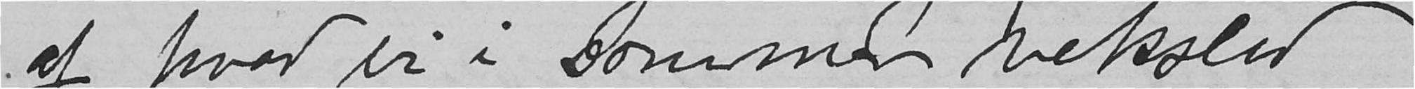af hvad vi i sommen veksled
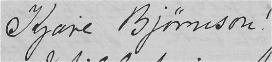Kjære Bjørnson!
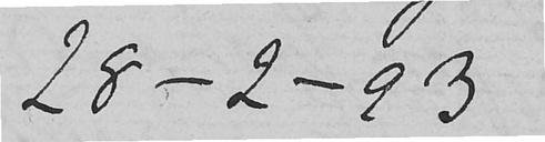28-2-93
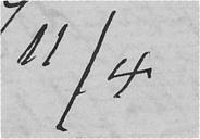11/4.
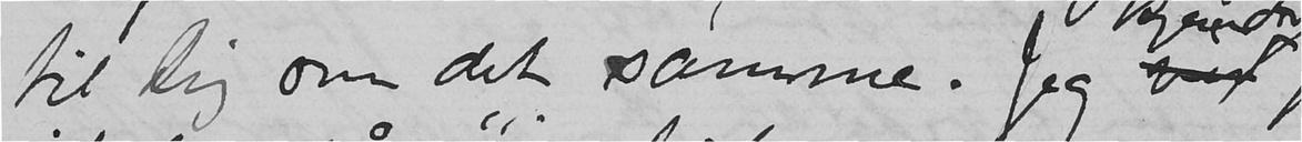til Dig om det samme. Jeg ved
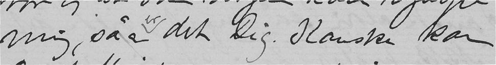mig, så er det Dig. Kanske kan
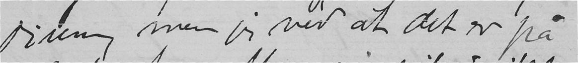dium, men jeg ved at det er på
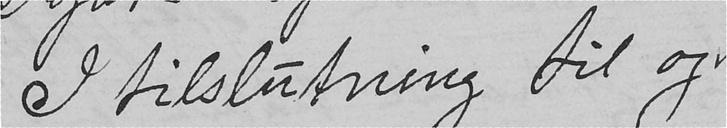I tilsluting til og
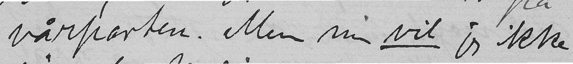vårparten. Men nu vil jeg ikke
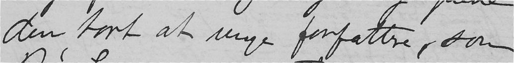den tort at unge forfattere, som
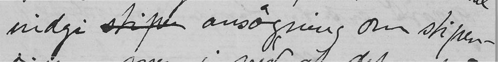indgi stipen ansøgning om stipen-
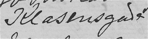Klasensgade
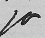jo
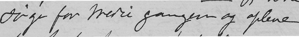søge for tredie gangen og opleve
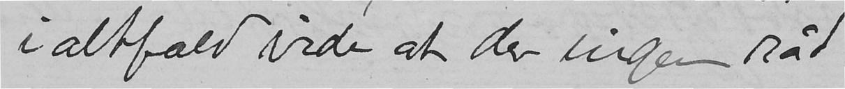i altfald vide at der ingen råd
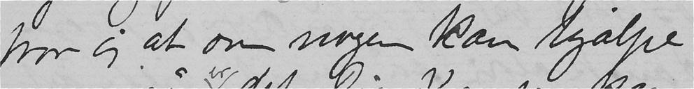tror jeg at om nogen kan hjælpe
